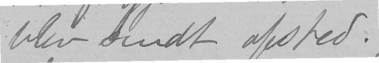blev sendt afsted.
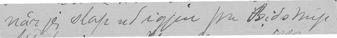når jeg slap ud igjen fra Bidstrup.
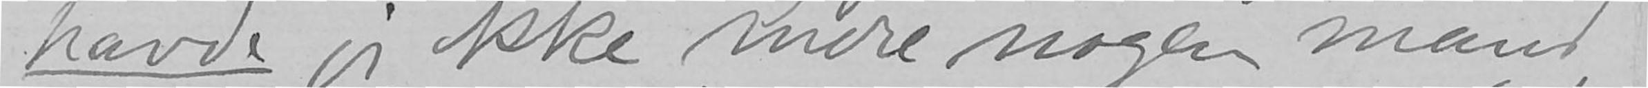havde jeg ikke mere nogen mand
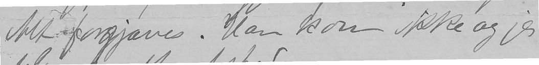Alt forgjæves. Han kom ikke og jeg
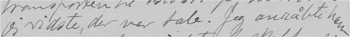jeg vidste, der var tale. Jeg anråbte ham
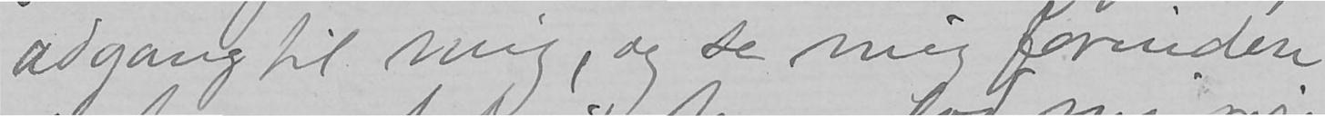adgang til mig, og se mig forinden,
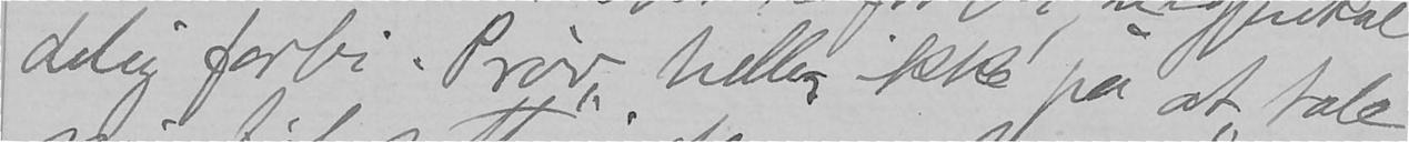delig forbi. Prøv heller ikke på at "tale
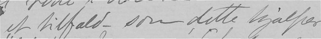et tilfælde som dette hjælper
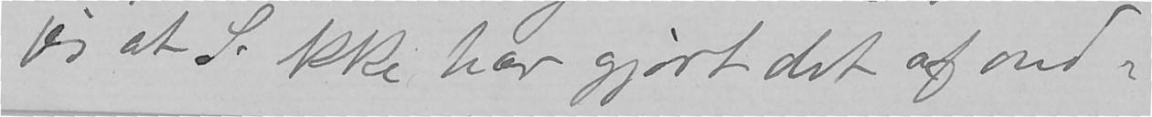jeg at S. kke har gjort det af ond-
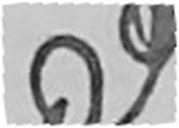I
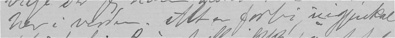her i verden. Alt er forbi, uigjenkal
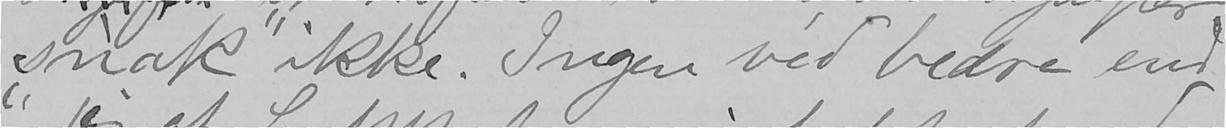"snak" ikke. Ingen véd bedre end
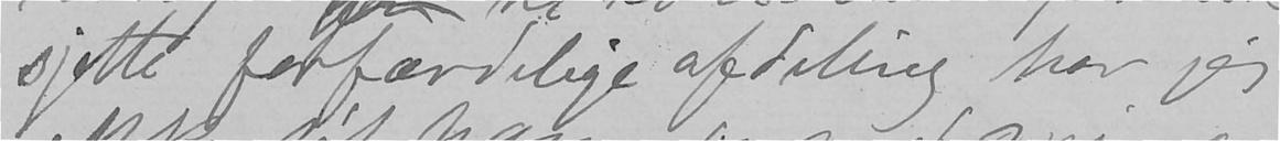sjette forfærdelige afdeling har jeg
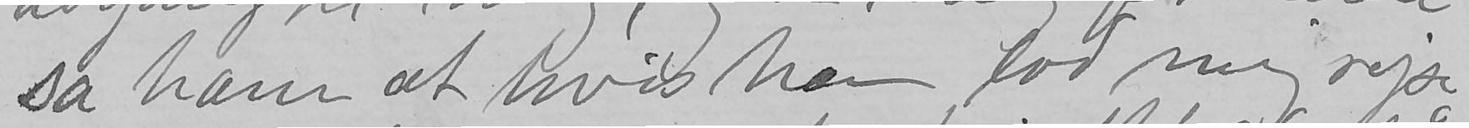sa ham at hvis han lod mig rejse
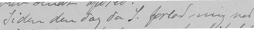Siden den dag da S. forlod mig ved
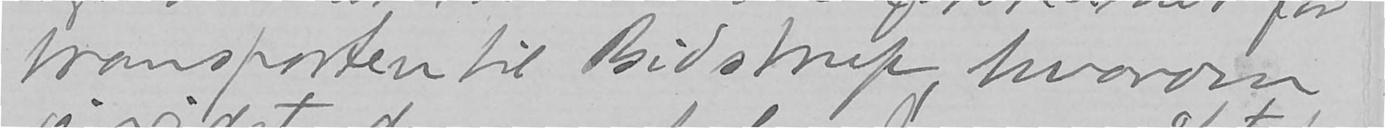transporten til Bidstrup, hvorom
